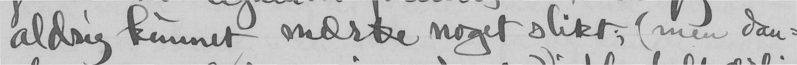aldrig kunnet mærke noget slikt, (men dan-
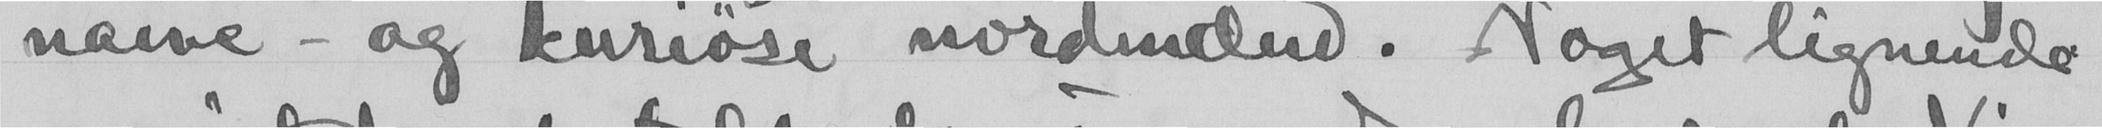naive - og kuriøse nordmænd. Noget lignende
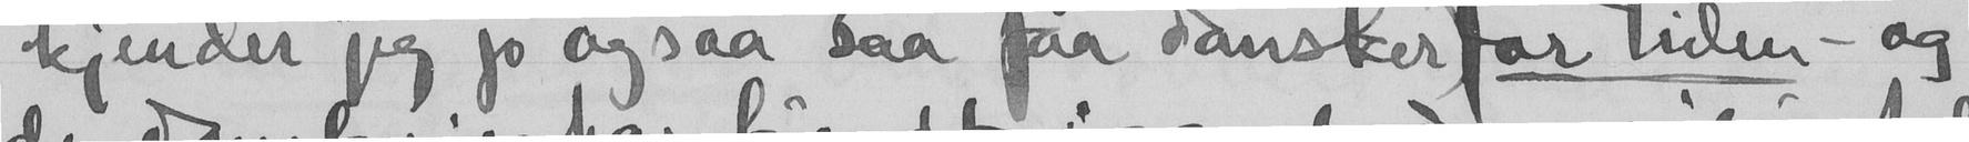kjender jeg jo ogsaa saa faa dansker for tiden - og
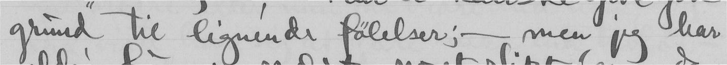grund til lignende følelser; - men jeg har
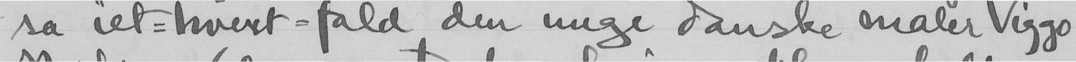sa iet-hvert -fald den unge danske maler Viggo
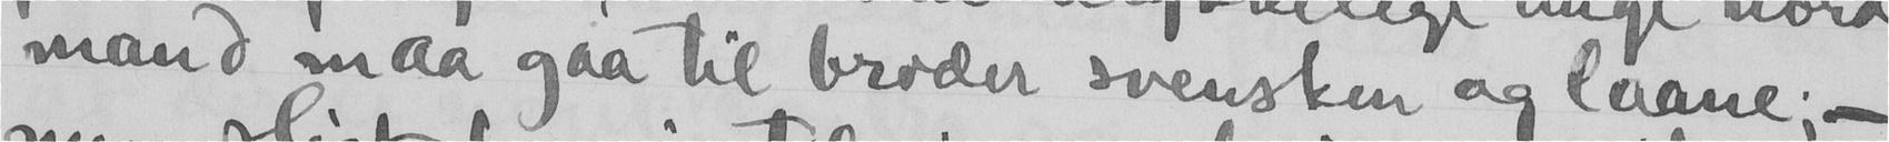mand maa gaa til broder svensken og laane; -
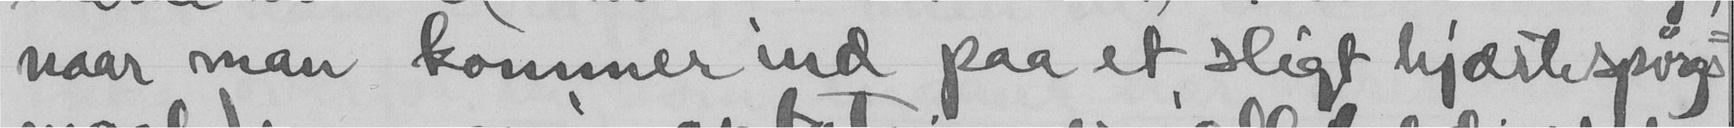naar man kommer ind paa et sligt hjærtespørgs-
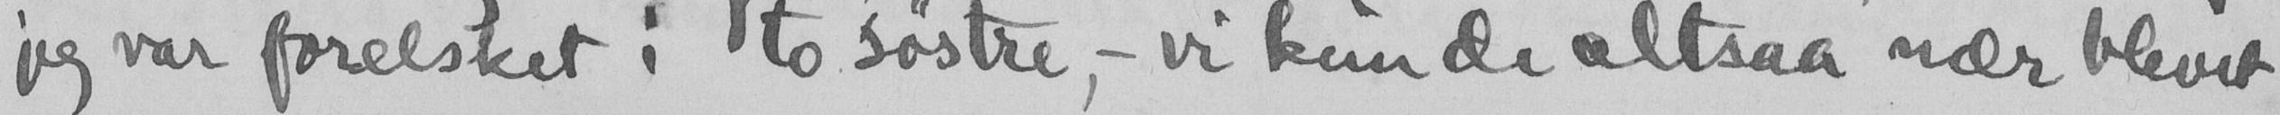jeg var forelsket i to søstre, - vi kunde altsaa nær blevet
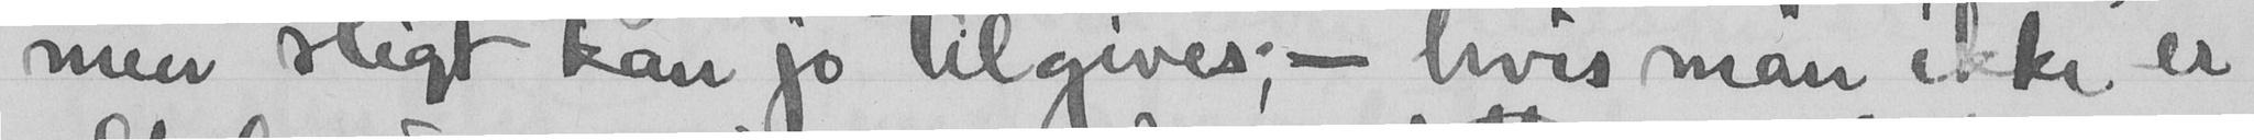men sligt kan jo tilgives, - hvis man ikke er
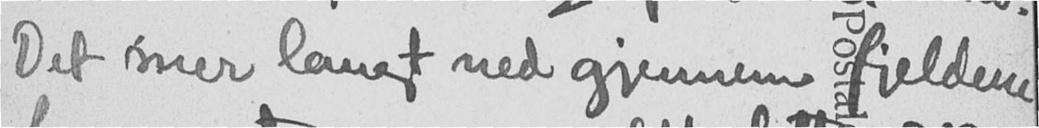Det sner langt ned gjennem fjeldene
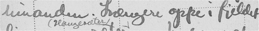hinanden. Længere oppe i fjeldet
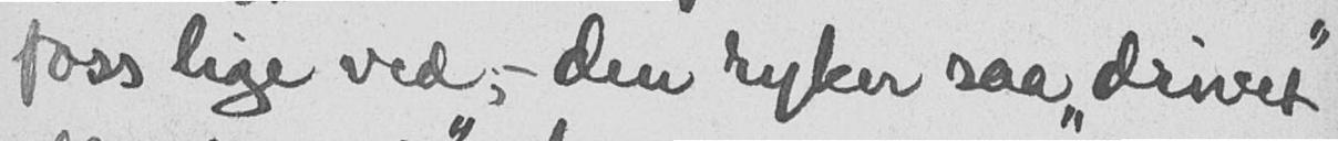foss lige ved, - den ryker saa "drivet"
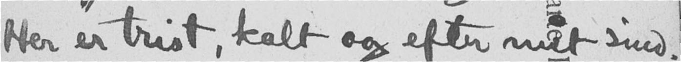Her er trist, kalt og efter mit sind.
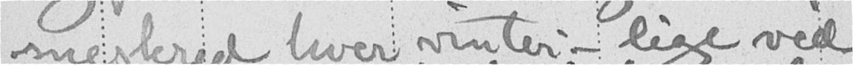sneskred hver vinter - lige ved
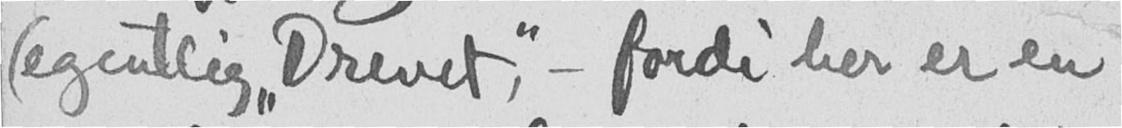(egentlig "Drevet" - fordi her er en
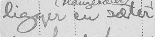ligger en sæter
